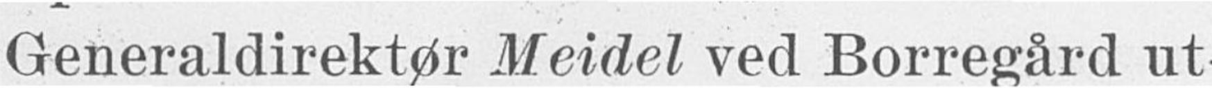Generaldirektør Meidel ved Borregård ut-
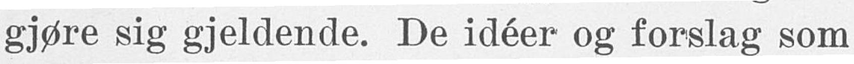gjøre sig gjeldende. De idéer og forslag som
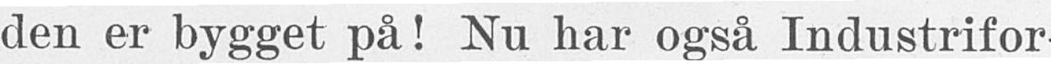den er bygget på! Nu har også Industrifor-
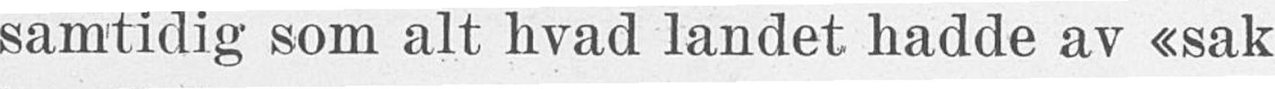samtidig som alt hvad landet hadde av "sak-
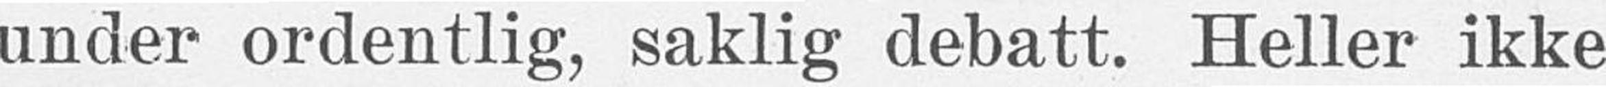under ordentlig, saklig debatt. Heller ikke
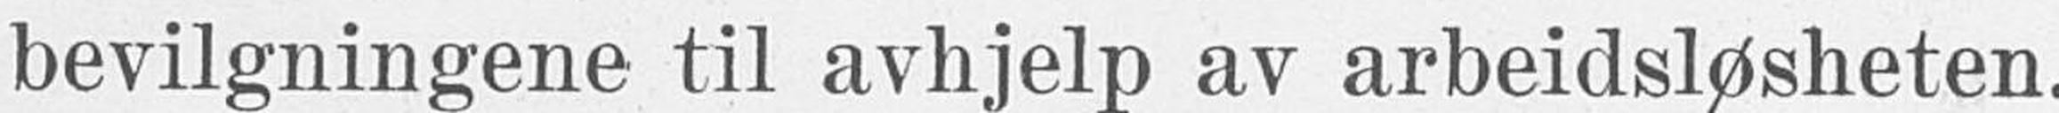bevilgningene til avhjelp av arbeidsløsheten.
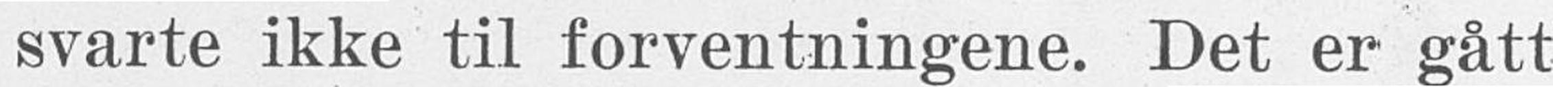svarte ikke til forventningene. Det er gått
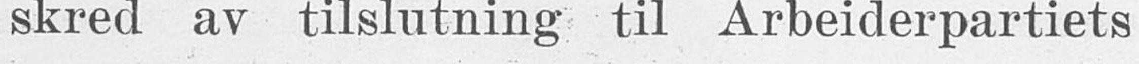skred av tilslutning til Arbeiderpartiets
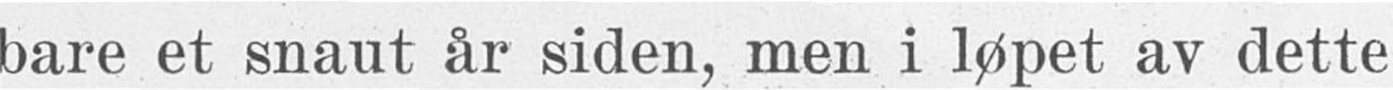bare et snaut år siden, men i løpet av dette
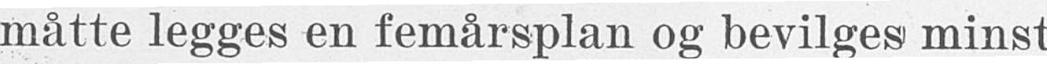måtte legges en femårsplan og bevilges minst
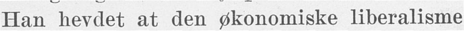Han hevdet at den økonomiske liberalisme
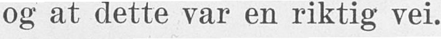og at dette var en riktig vei.
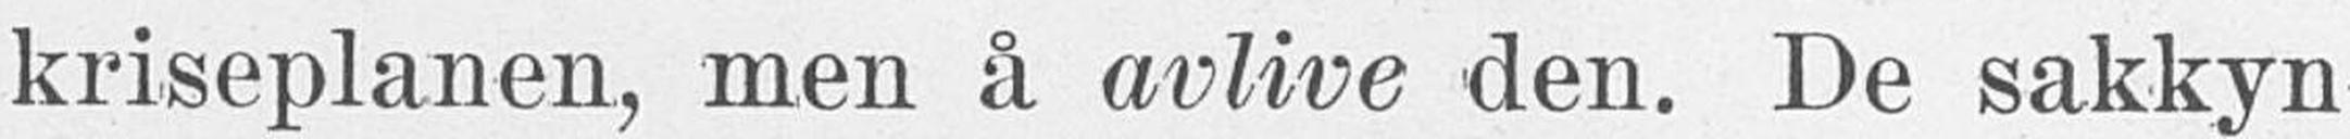kriseplanen, men å avlive den. De sakkyn-
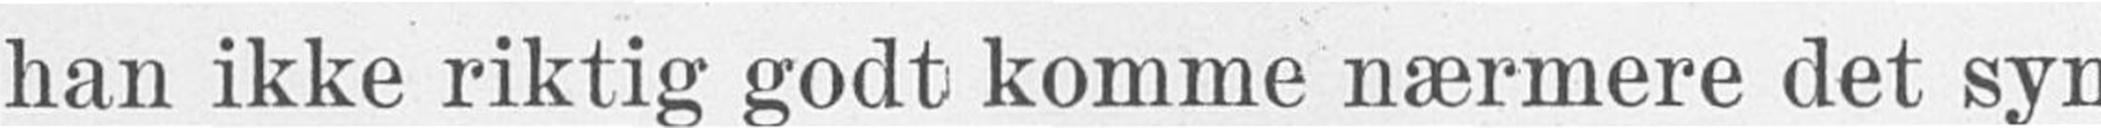han ikke riktig godt komme nærmere det syn
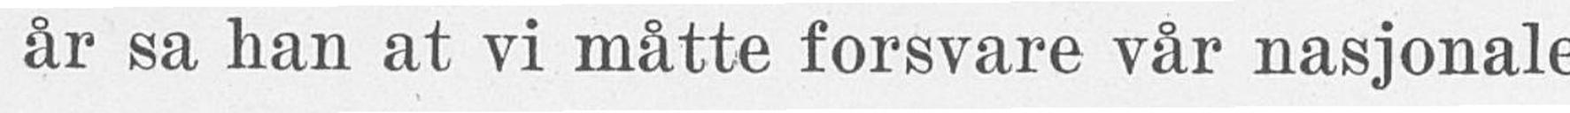år sa han at vi måtte forsvare vår nasjonale
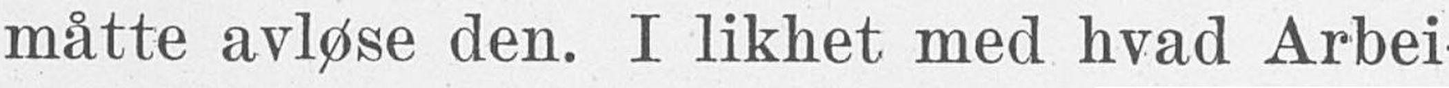måtte avløse den. I likhet med hvad Arbei
